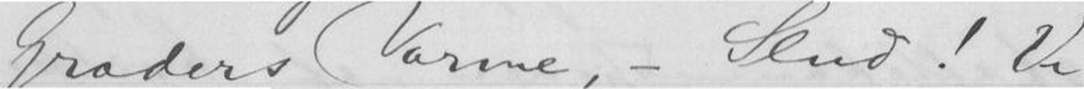Graders Varme, - Slud! Vi
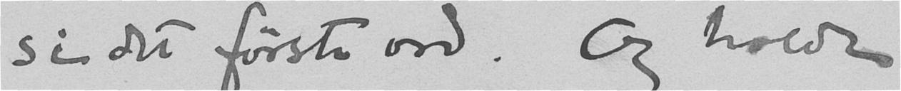si det første ord. Og holde
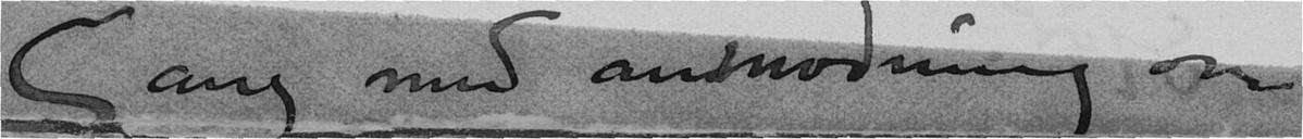Gang med andmodning om
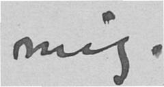mig.
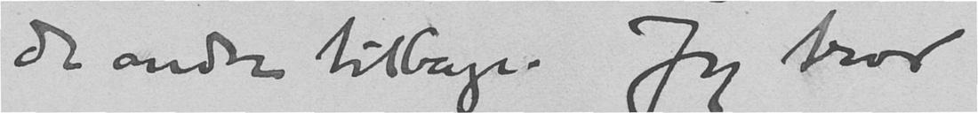de andre tilbage. Jeg tror
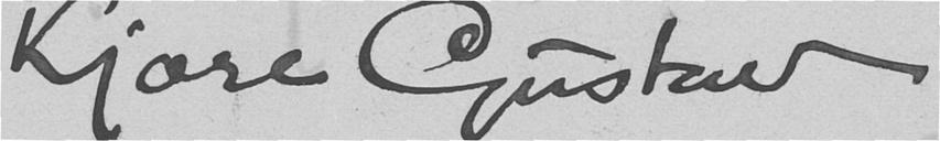Kjære Gustav
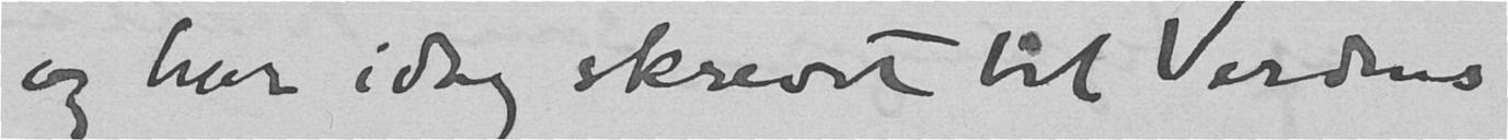og har idag skrevet til Verdens
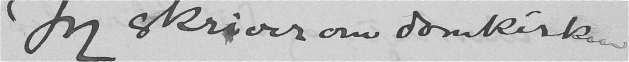Jeg skriver om domkirken
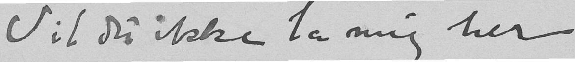Vil du ikke la mig her
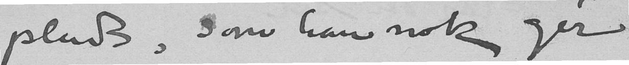plads, som han nok gir
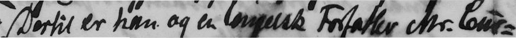Dertil er han og en engelsk Forfatter Mr. Cur-
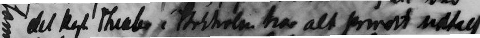det kgl Theater i Stokholm har alt pomdet udtalt
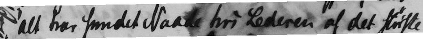alt har fundet Naade hos Lederen af det største
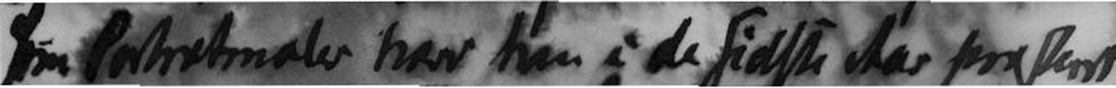som Portretmaler har han i de sidste Aar produseret
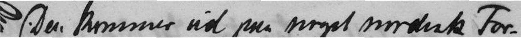Den kommer ud paa noget nordisk For-
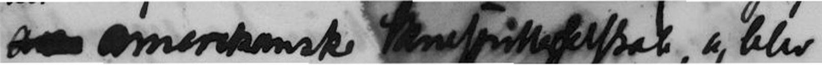amerikanske Skuespillerselskab, og blir
